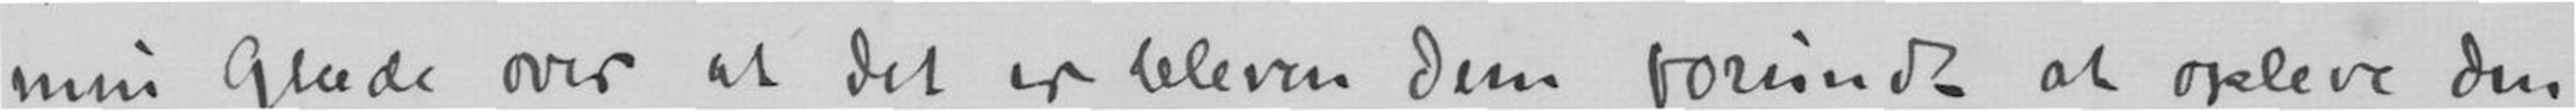min Glæde over at det er bleven Dem forundt at opleve den
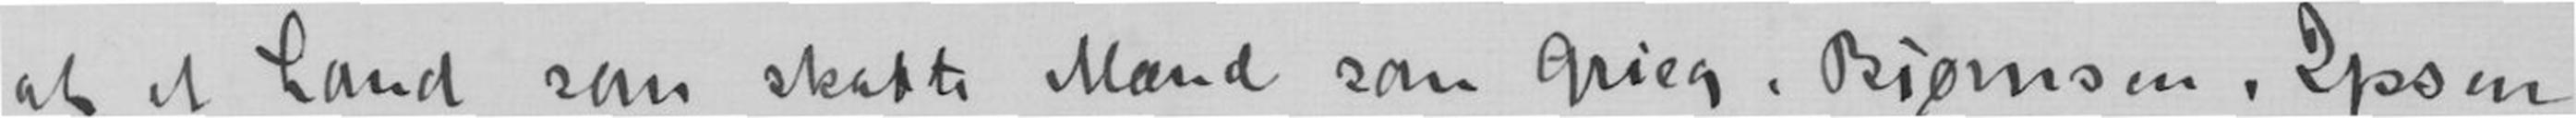at et Land som skabte Mænd som Grieg, Bjørnson, Ipsen
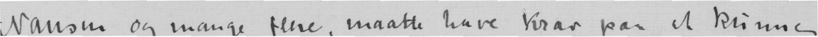Nansen og mange flere, maatte have krav paa at kunne
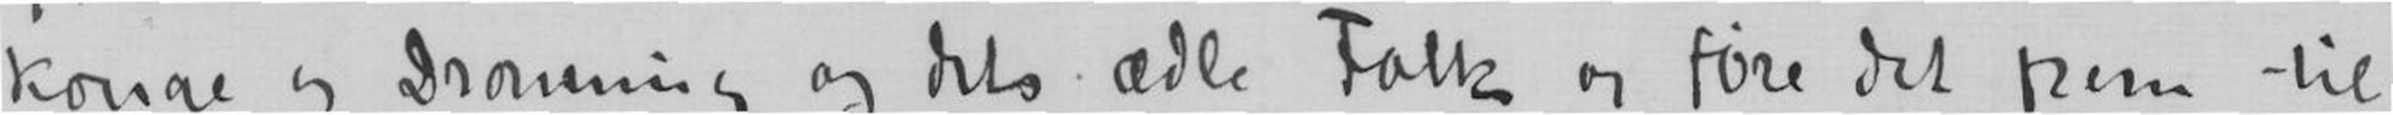Konge og Dronning og dets ædle Folk og føre det frem til
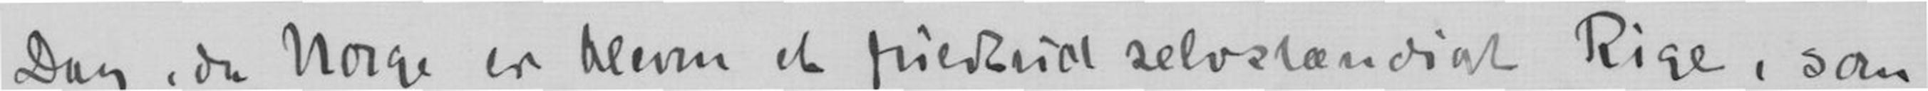dag, da Norge er bleven et friedtud selvstendigt Rige, som
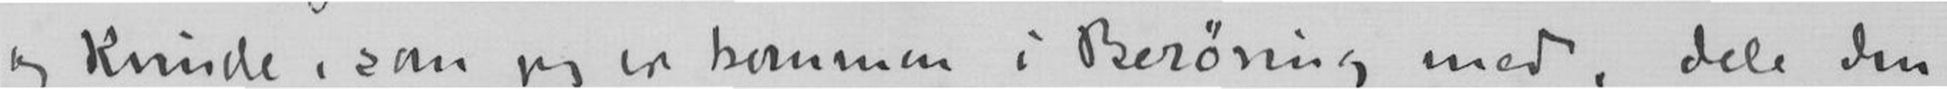og Kvinde, som jeg er kommen i Berøring med, dele den
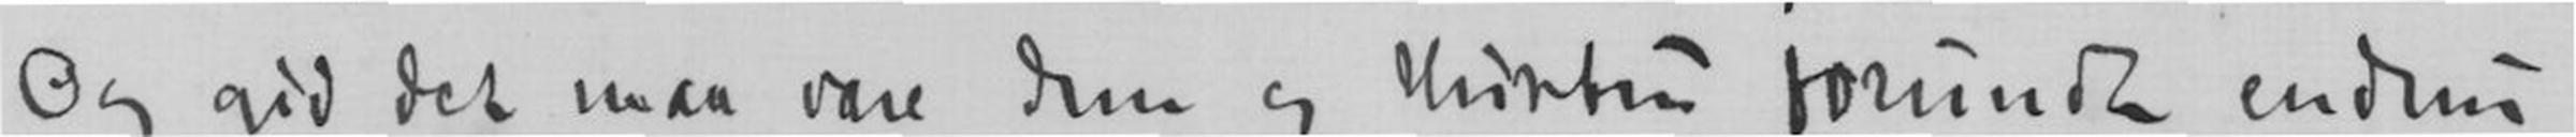Og gid det maa være Dem og Hustru forundt endnu
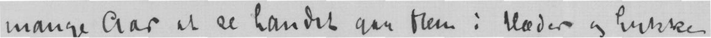mange Aar at se Landet gaa Dem i Hæder og Lykke
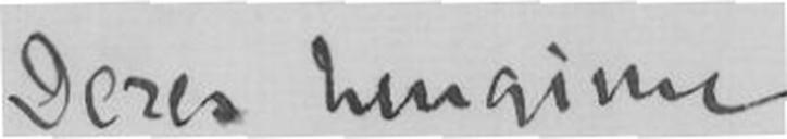Deres hengivne
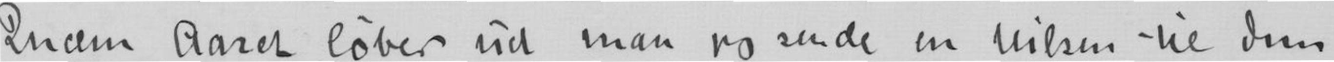Inden Aaret løber ud maa jeg sende en hilsen til Dem
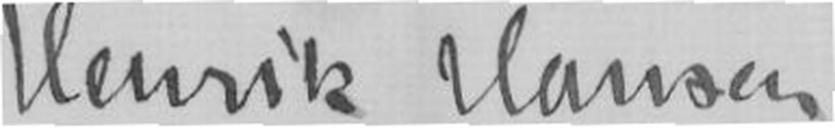Henrik Hansen
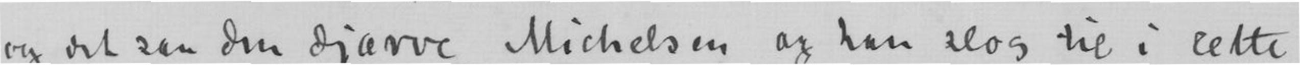og det saa den djerve Michelsen og han slog til i rette
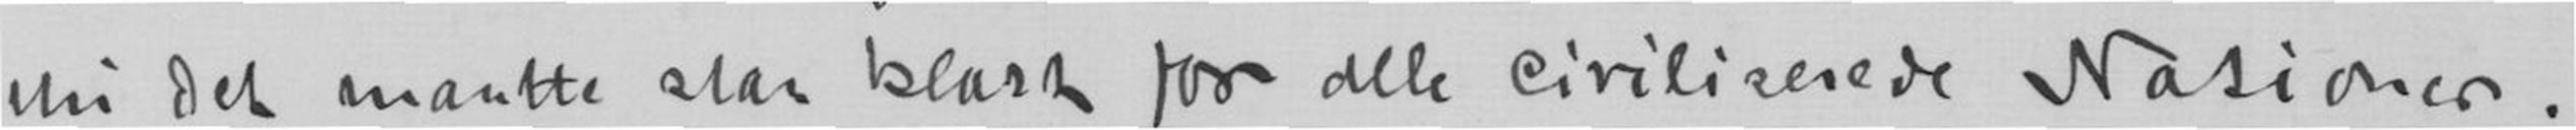thi det maatte staa klart for alle civiliserede Nationer
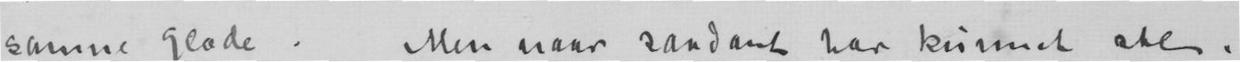samme Glæde. Men naar saadant har kunnet ske
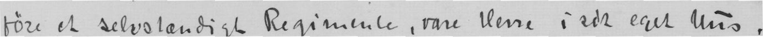føre et selvstendigt Regimente, være Herre i sit eget Hus,
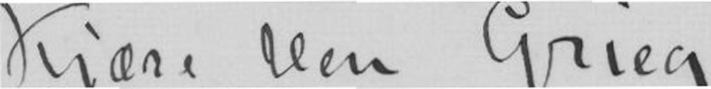Kjære Herr Grieg
